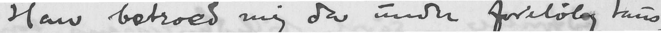Han betroed mig da under foreløbig taus-
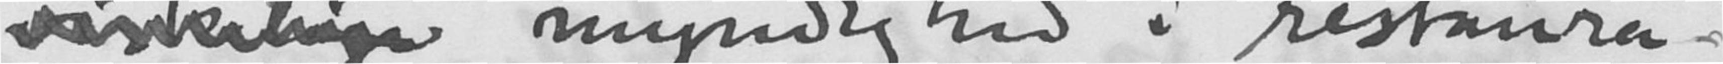virkelige myndighed i restaura-
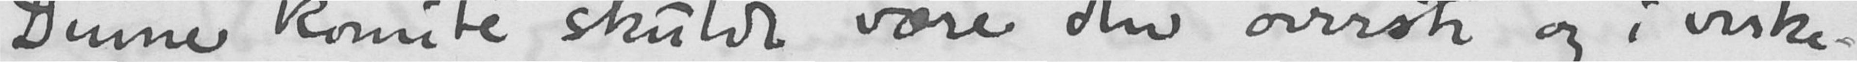Denne komité skulde være den øverste og i virke-
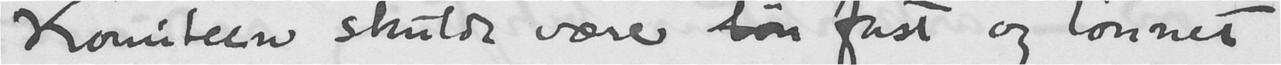Komiteen skulde være bør fast og lønnet
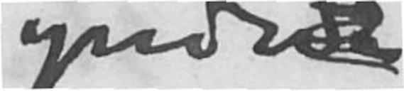ynde
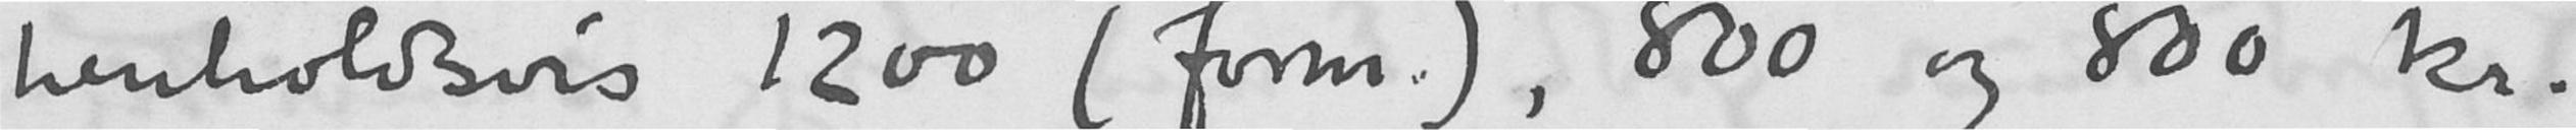henholdsvis 1200 (form.), 800 og 800 kr.
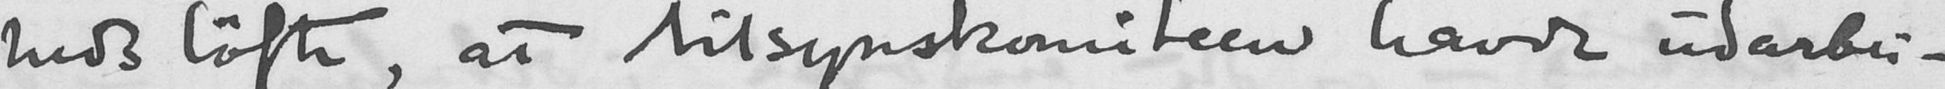hedsløfte, at tilsynskomiteen havde udarbei-
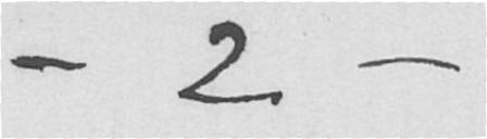- 2 -
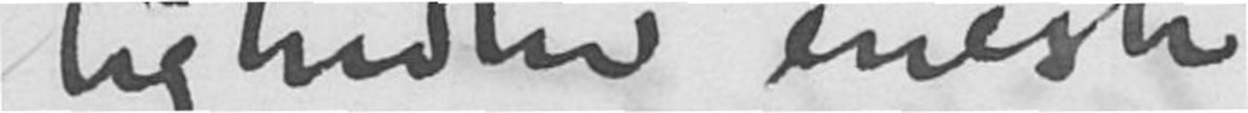ligheden eneste
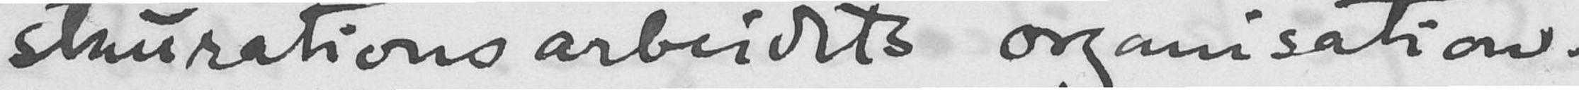staurationsarbeidets organisation.
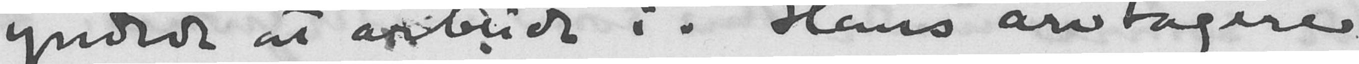yndede at arbeide i. Hans arvtagere
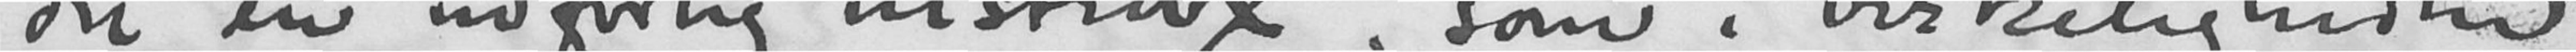det en udførlig "instrux", som i virkeligheden
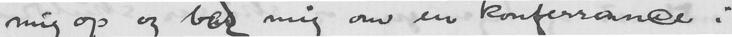mig op og bad mig om en konferrance i
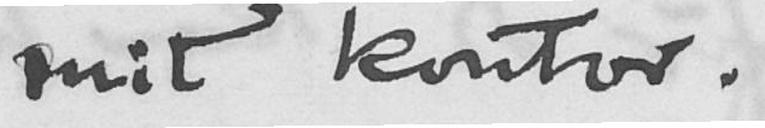mit kontor.
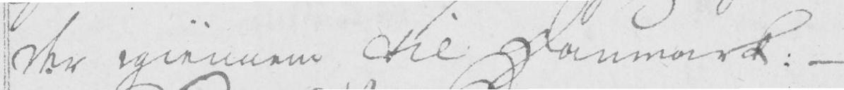der igiennem til Danmark. -
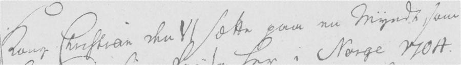Kong Christian den VI sætte paa en Myndt, som
